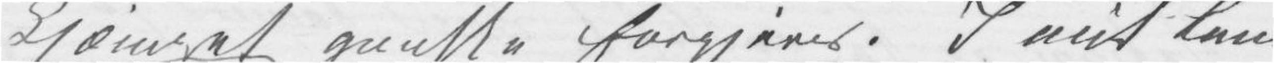kjæmpet ganske forgjeves. I mit Land
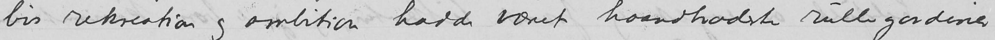livs rekreation og ambition hadde været haandbroderte rullegardiner
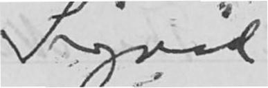Sigrid
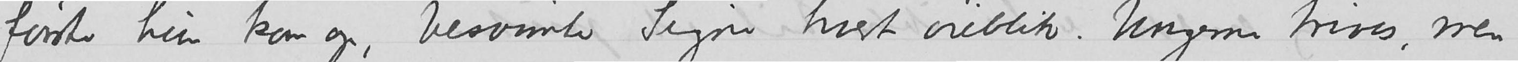første hun kom op, besvimte Signe hvert øieblik. Ungerne trives, men
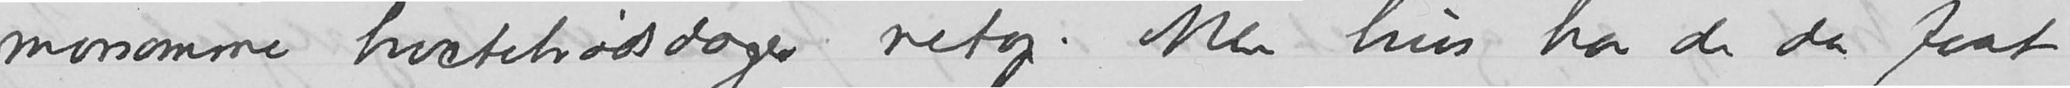morsomme hvætebrødsdager netop. Men hus har de da faat
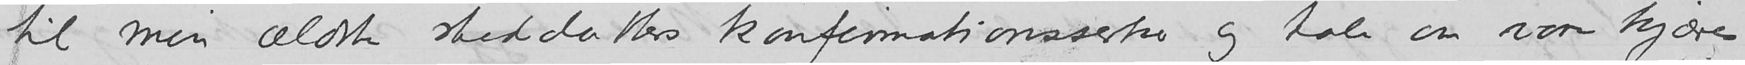til min ældste steddatters konfirmationsserker og tale om vore kjære
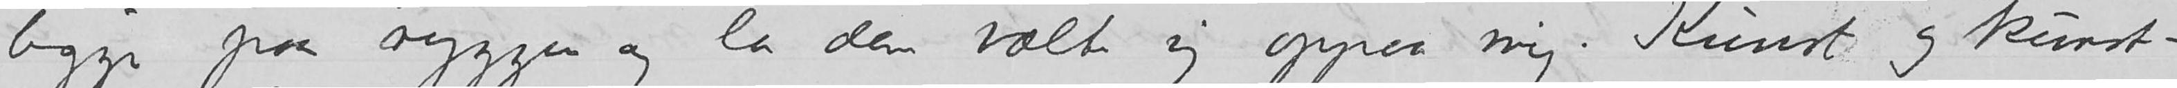ligge paa ryggen og la dem vælte sig oppaa mig. Kunst og kunst-
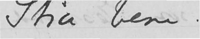Stia bene!
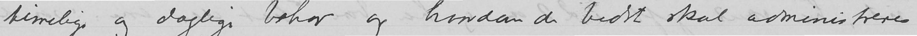timelige og daglige behov og hvordan de bedst skal administreres
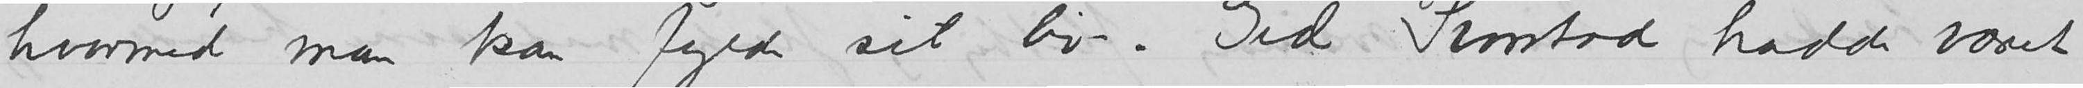hvormed man kan fylde sit liv. Gid Svarstad hadde været
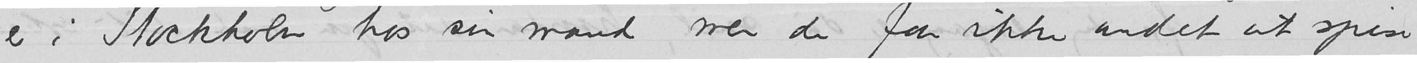er i Stockholm hos sin mand men de faar ikke andet at spise
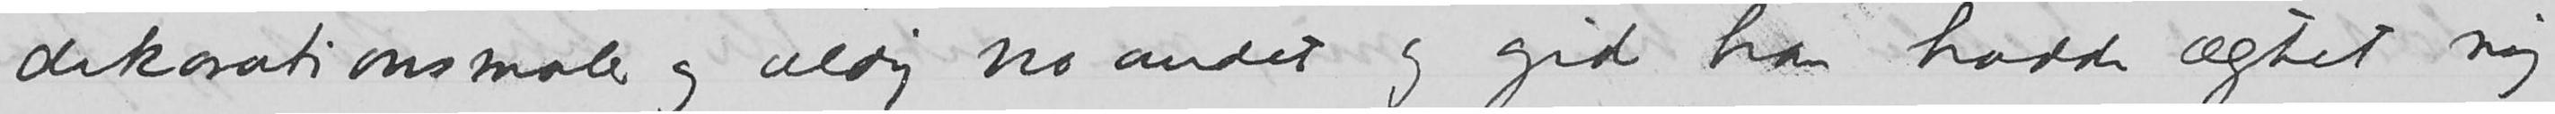dekorationsmaler og aldrig no andet og gid han hadde ægtet mig
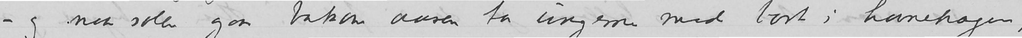– og naar solen gaar bakom aasen ta ungerne med bort i havnehagen,
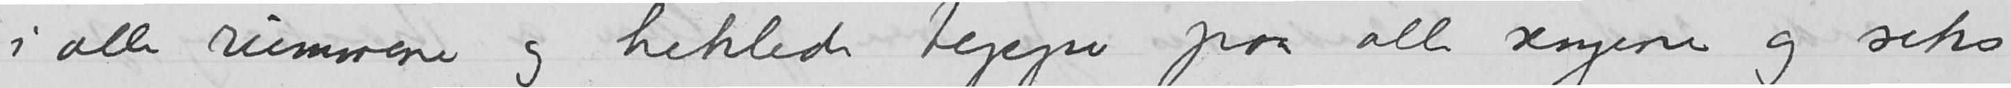i alle rummene og heklede tepper paa alle sengene og seks
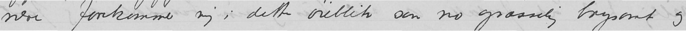nere forekommer mig i dette øieblik som no græsselig brysomt og
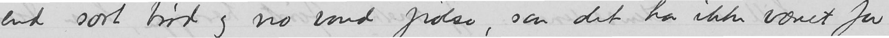end sort brød og no vond pølse, saa det har ikke været  for
